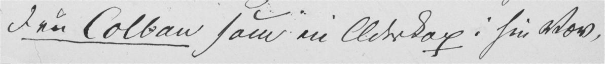Fru Colban som en Æderkop i sin Væv,
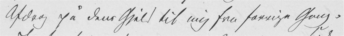Afdrag på dens Gjeld til mig fra forrige Gang.
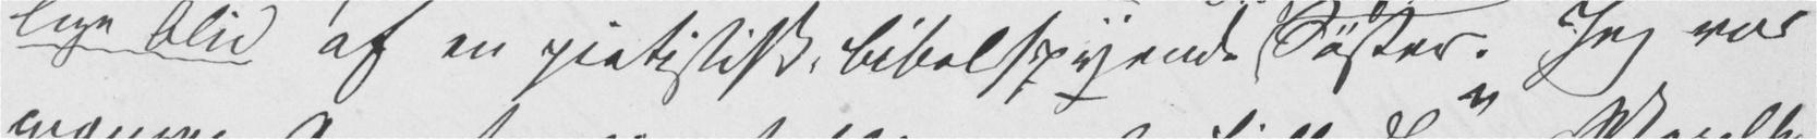lige blid af en pietistisk, bibelspyende Søster. Jeg var
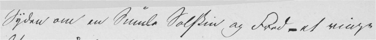Syden om en Smule Solskin og Fred – et ringe
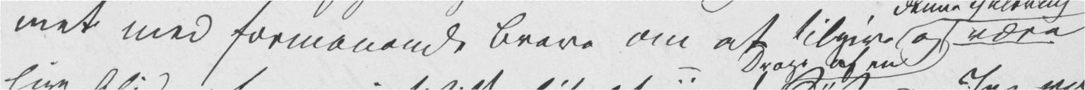met med formanende Breve om at tilgive og være
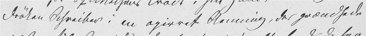Frøken Schreiber i en opirret Stemning, der grændsede
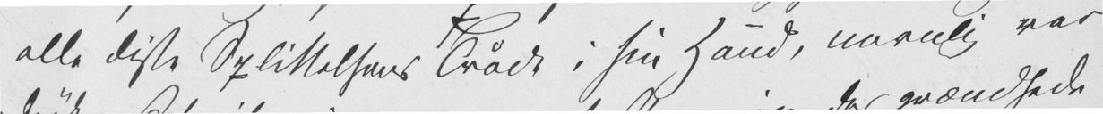alle disse Splittelsens Tråde i sin Hand, navnlig var
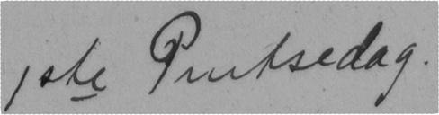1ste Pintsedag.
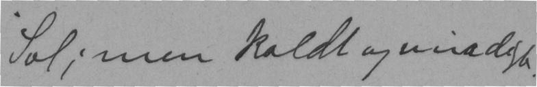Sol; men kaldt og vindigt.
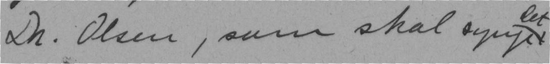Dr. Olsen, som skal synge
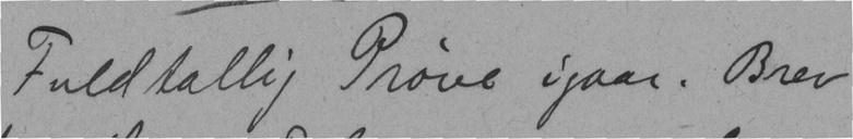Fuldtallig Prøve igaar. Brev
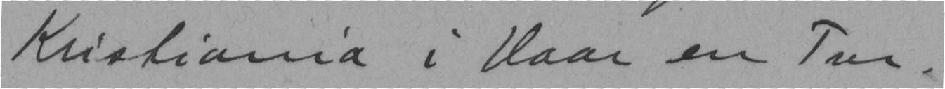Kristiania i Vaar en Tur.
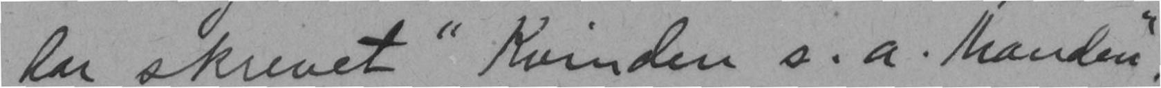har skrevet "Kvinden s. a. Manden".
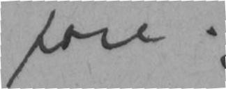føre.
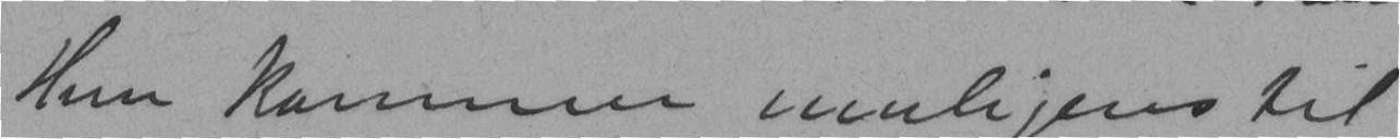Hun kommer muligens til
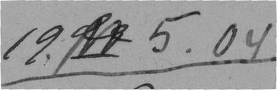19. 5.04
